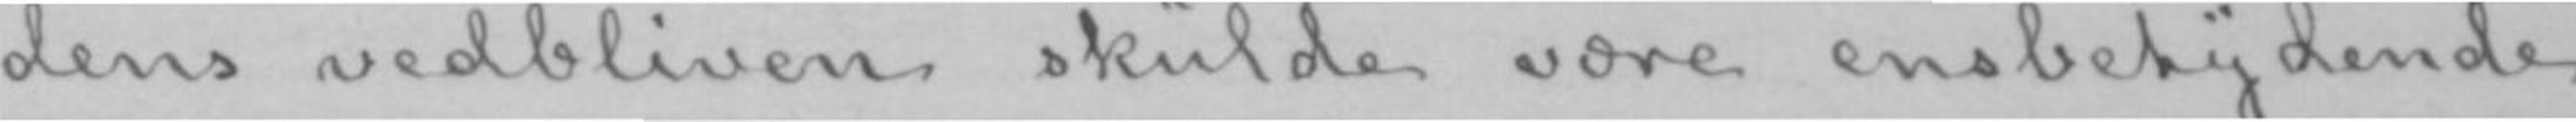dens vedbliven skulde være ensbetydende
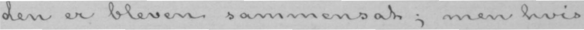den er bleven sammensat; men hvis
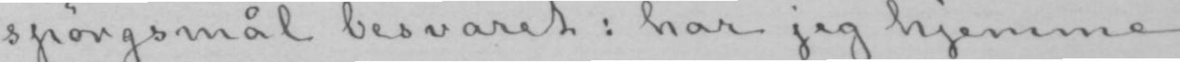spørgsmål besvaret: har jeg hjemme
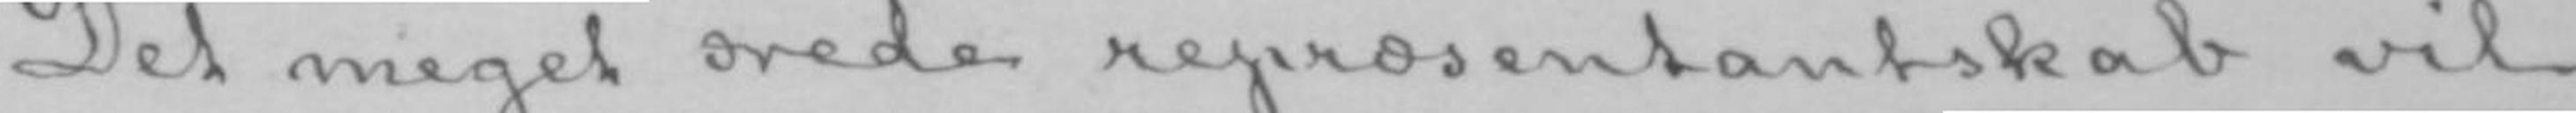Det meget ærede repræsentantskab vil
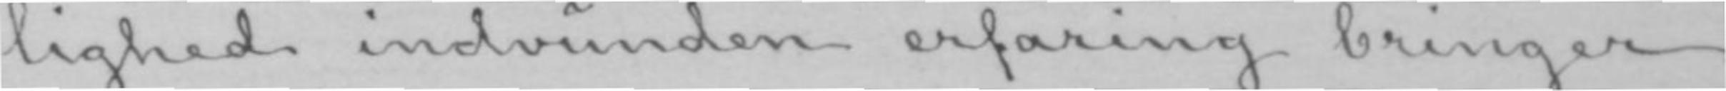lighed indvunden erfaring bringer
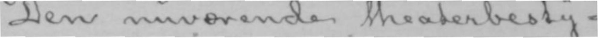Den nuværende theaterbesty-
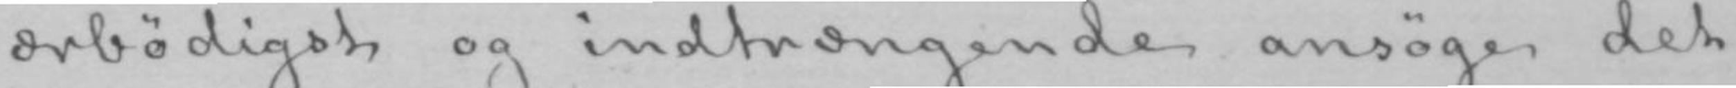ærbødigst og indtrængende ansøge det
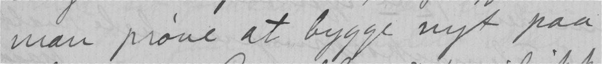man prøve at bygge nyt paa
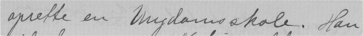oprette en Ungdomsskole. Han
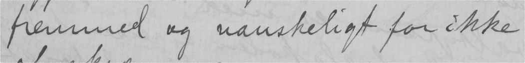fremmed og vanskeligt for ikke
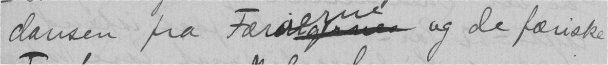dansen fra Fær og de færiske
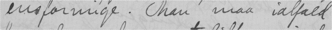ensformige. Man maa ialfald
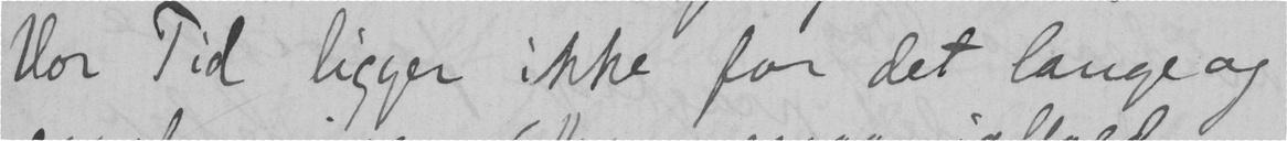Vor Tid ligger ikke for det lange og
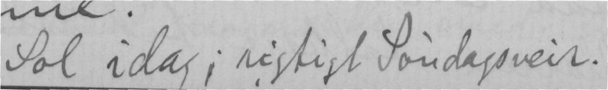Sol idag; rigtigt Søndagsveir.
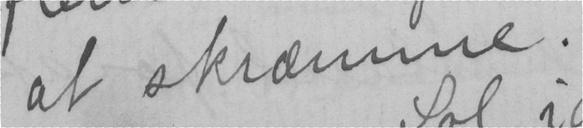at skræmme.
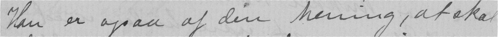Han er ogsaa af den mening, at skal
